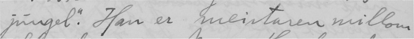jungel". Han er meistaren millom
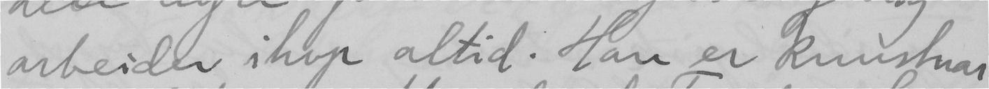arbeider ihop altid. Han er kunstnar
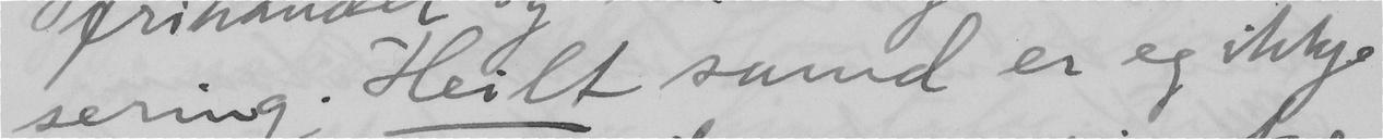sering. Heilt samd er eg ikkje
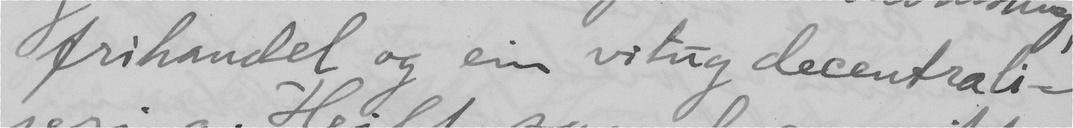frihandel og ein vitug decentrali-
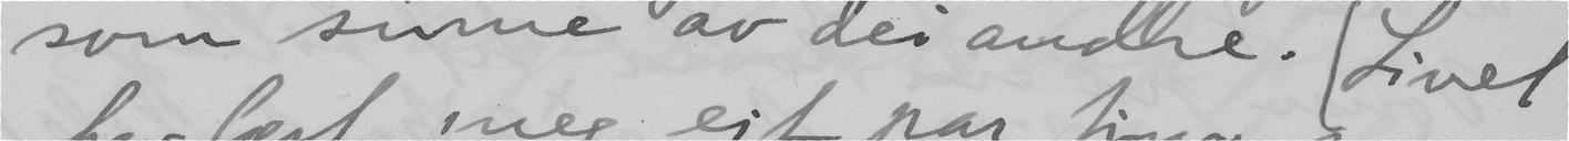som sume av dei andre. (Livet
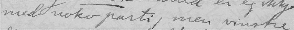med noko parti, men vinstre
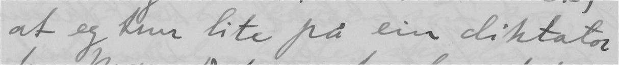at eg trur lite på ein diktator
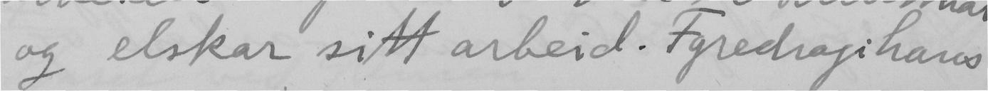og elskar sitt arbeid. Fyredragi hans
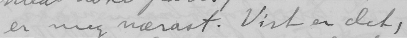er meg nærast. Vist er det,
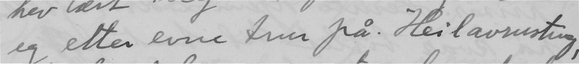eg etter evne trur på. Heilavrusting,
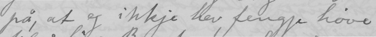på, at eg ikkje hev fengje höve
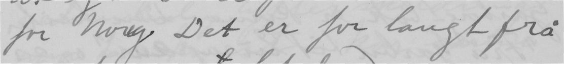for Noreg. Det er for langt frå
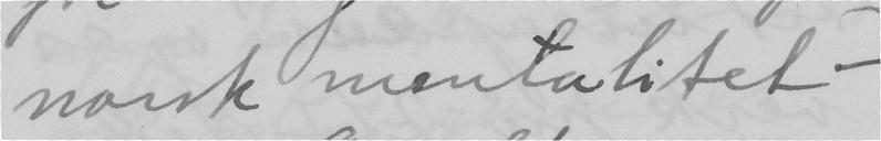norsk mentalitet –
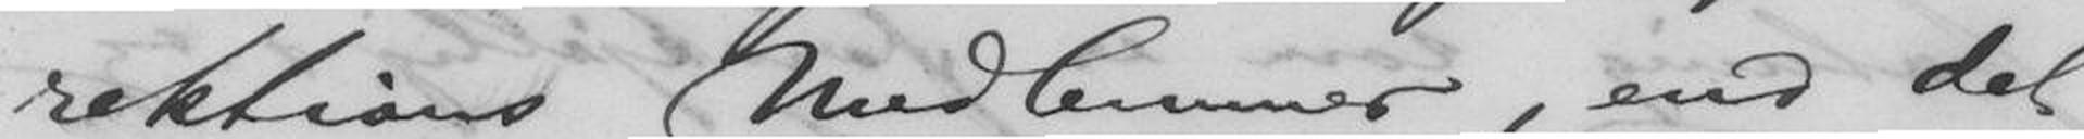rektions Medlemmer, end det
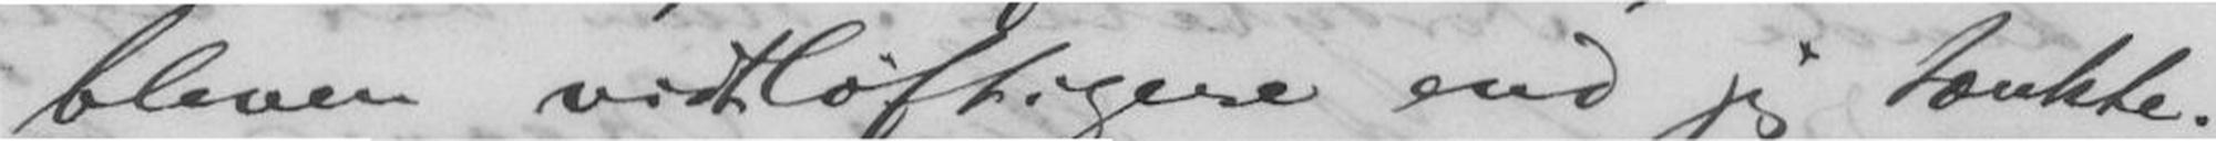bleven vidtløftigere end jeg tænkte.
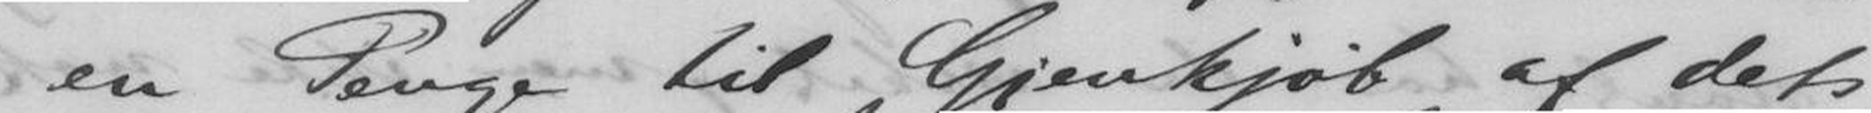en Penge til Gjenkjøb af dets
